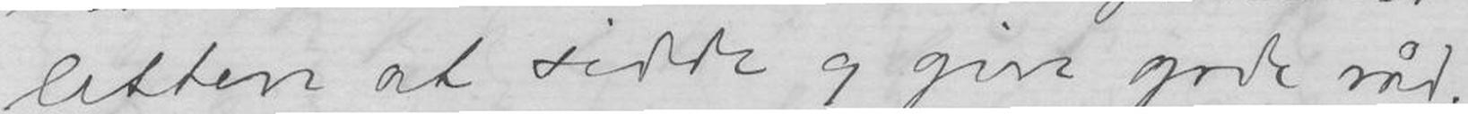lettere at sidde og give gode råd.
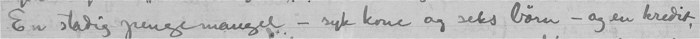En stadig pengemangel - syk kone og seks børn - og en kredit,
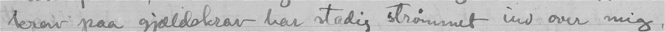krav paa gjældskrav har stadig strømmet ind over mig,
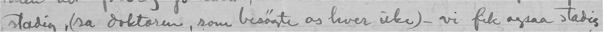stadig, (sa doktoren, som besøgte os hver uke), - vi fik ogsaa stadig
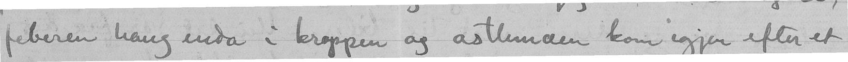feberen hang enda i kroppen og astmaen kom igjen efter et
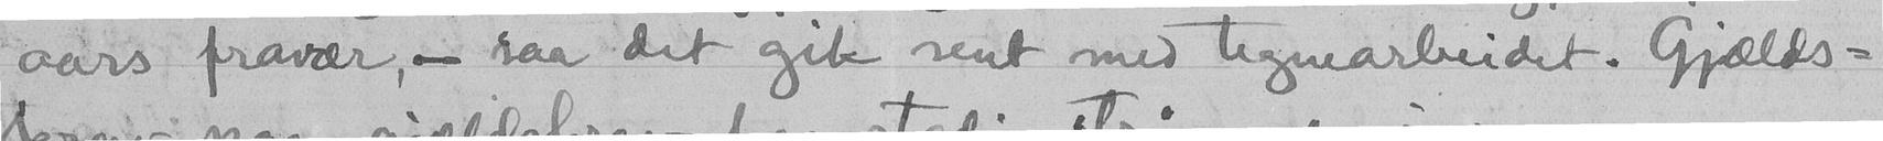aars- fravær, - saa  det gik sent med tegnearbeidet. Gjælds-
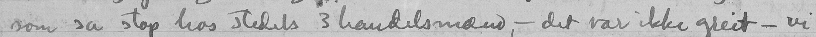som sa stop hos stedets 3 handelsmænd, - det var ikke greit - vi
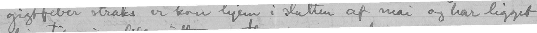gigtfeber straks vi kom hjem i slutten af mai og har ligget
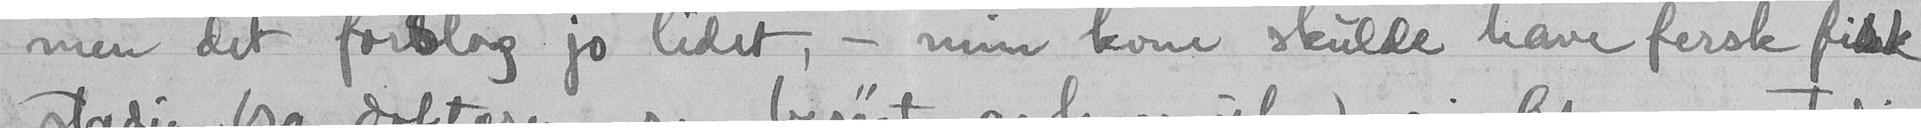men det forslog jo lidet, - min kom skulde have fersk fisk
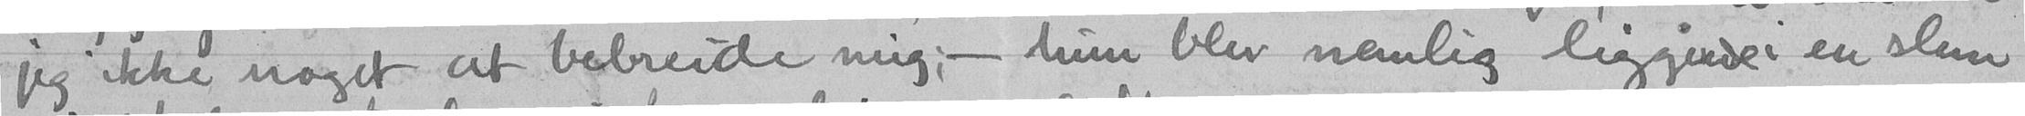jeg ikke noget at bebreide mig; - hun blev nemlig liggende en stund
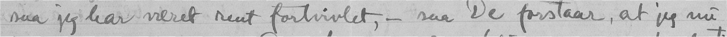saa jeg har været rent fortvivlet, - saa De forstaar, at jeg nu
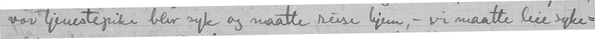vor tjenestepike blev syk og maatte reise hjem, - vi maatte leie syke-
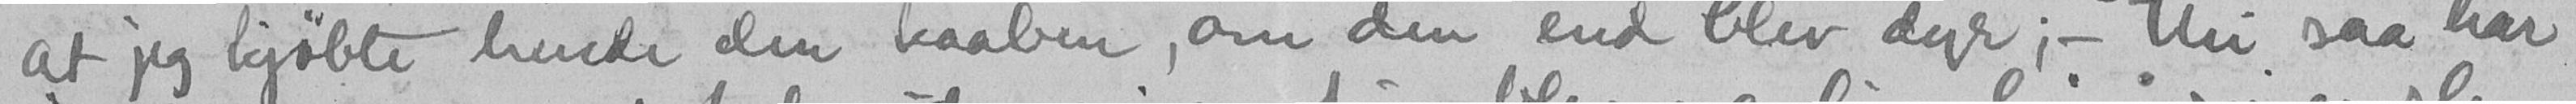at jeg kjøbte hende den kaaben, om den end blev dyr; - thi saa har
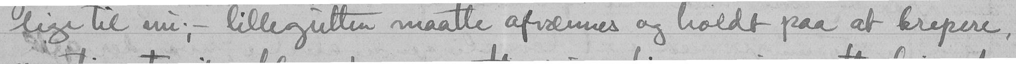lige til nu; - lillegutten maatte afvænnes og holdt paa at krepere,
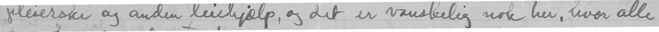pleierske og anden leiehjælp, og det er vanskelig nok her, hvor alle
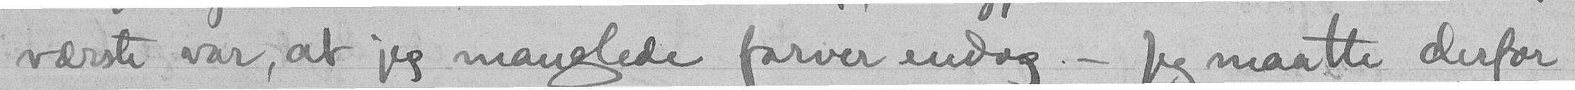værste var, at jeg manglede farver endog. - Jeg maate derfor
